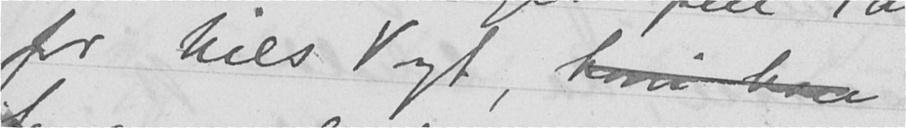for Nils Vogt, hvori han
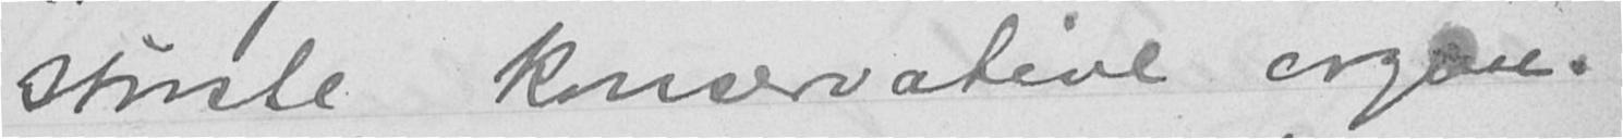største konservative organ.
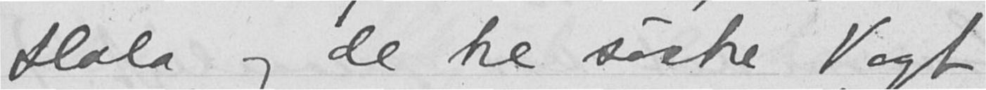Hola og de tre søstre Vogt
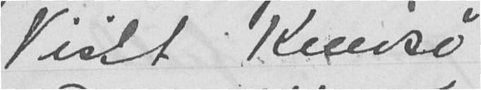Visitt Knivsø.
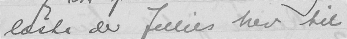læste der Julies brev til
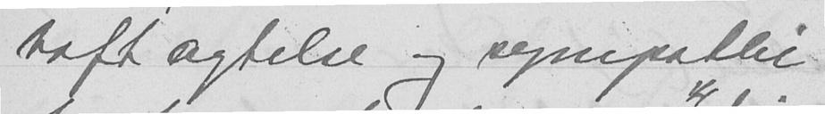haft agtelse og sympathi
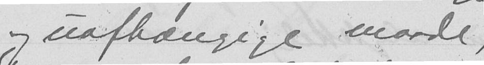og uafhængige maade,
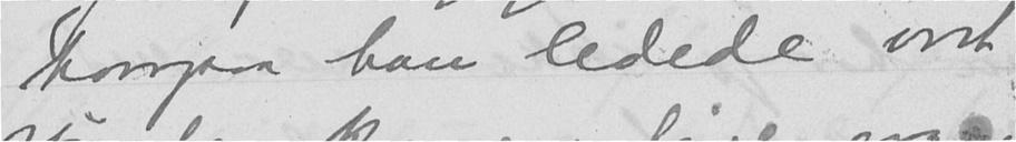hvorpaa han ledede vort
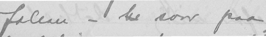Johan - be svar paa
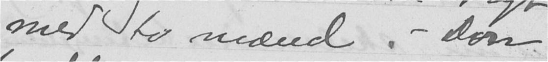med to mænd. - Don
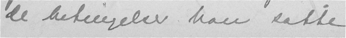de betingelser han satte
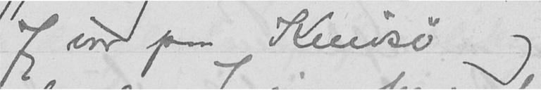Jeg var paa Knivsø og
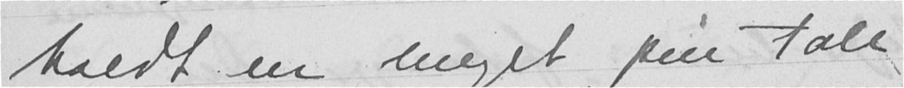holdt en meget pen tale
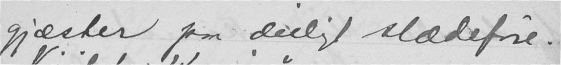gjæster paa deiligt slædeføre.
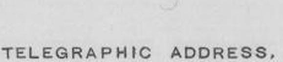TELEGRAPHIC ADDRESS.
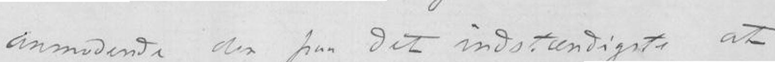anmodende der paa det indstændigste at
